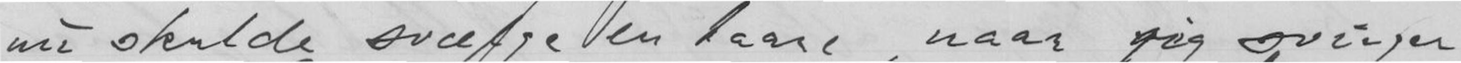nu skulde svælge en taare, naar jeg svinger
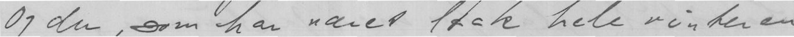Og du, som har været laak hele vinteren
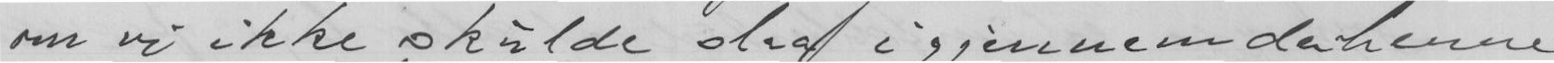om vi ikke skulde slaa igjennem derhenne
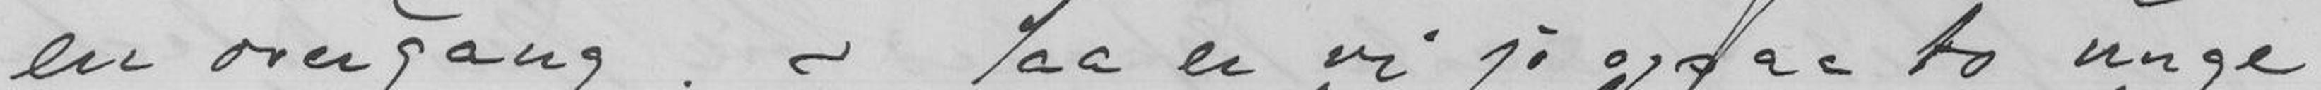en overgang. - Saa er vi jo ogsaa to unge
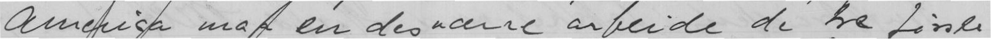America maa en desværre arbeide de tre første
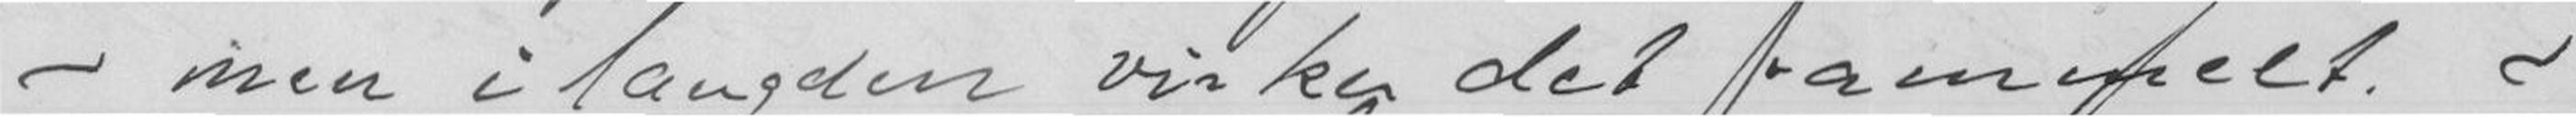- men ilængden virker det vammelt. -
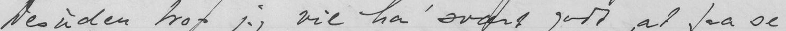Desuden tror jeg vil ha svært godt at faa se
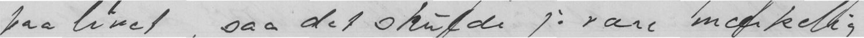paa livet, saa det skulde jo være mærkelig
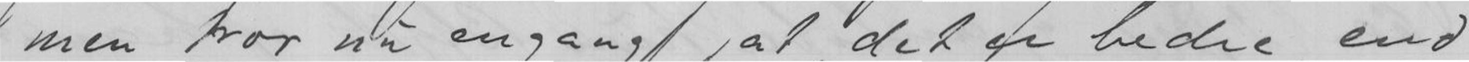men tror nu engang at det er bedre, end
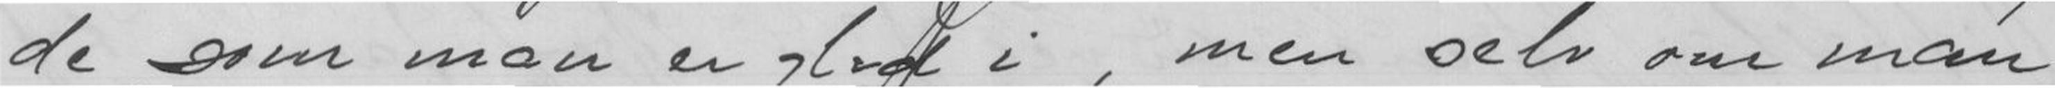de som man er glad i, men selv om man
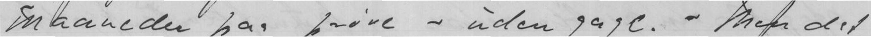Maaneder paa prøve - uden gage. - Men det
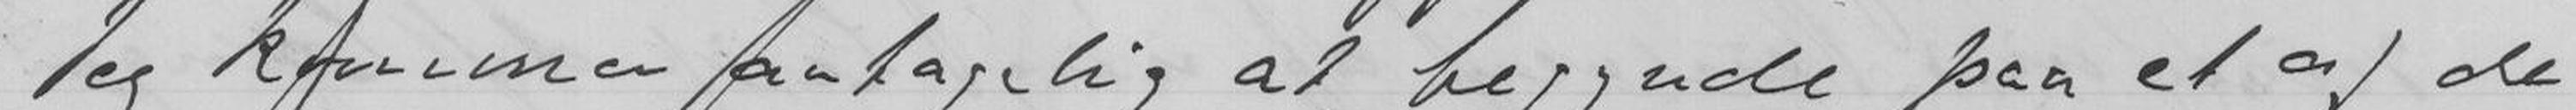- Jeg kommer antagelig at begynde paa et af de
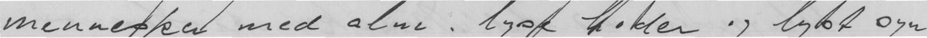mennesker med alm. lyse hoder og lyst syn
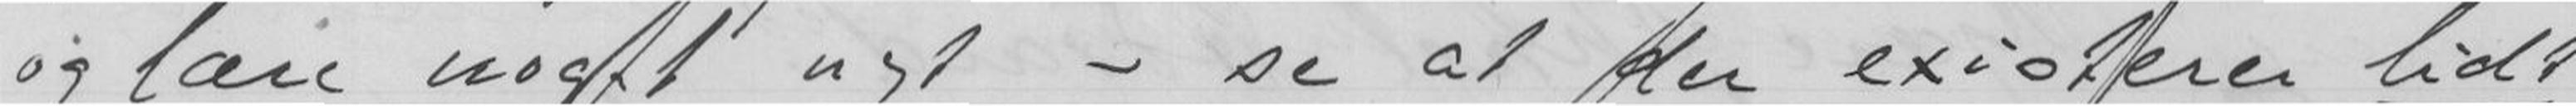og lære noget nyt - se at der existerer lidt
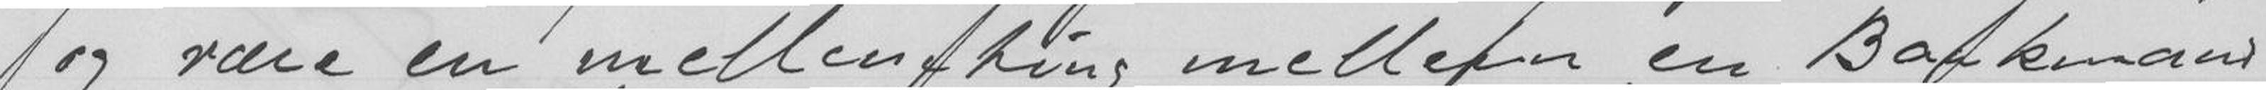og være en mellemting mellem en Bankmand
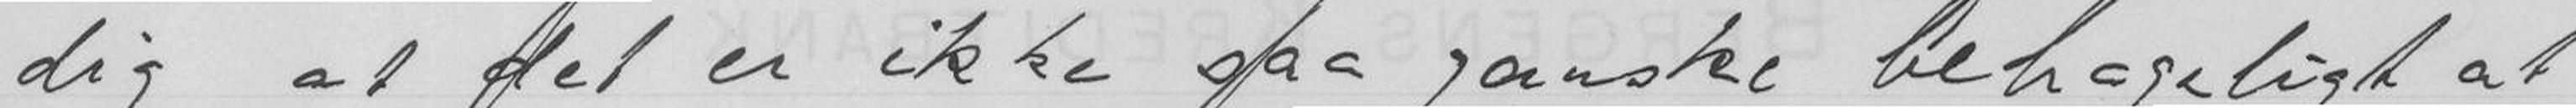dig, at det er ikke ganske behageligt at
